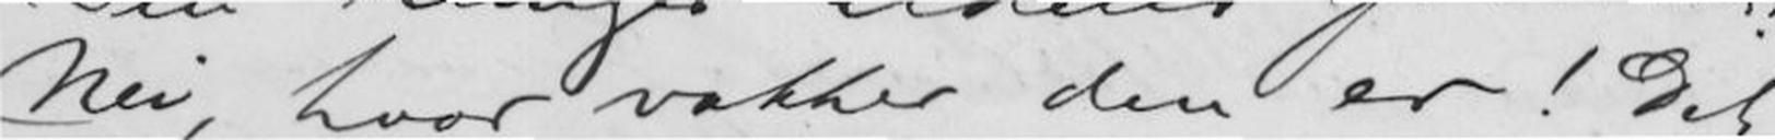Nei, hvor vakker den er! Det
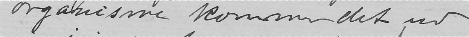organisme kommer det ud
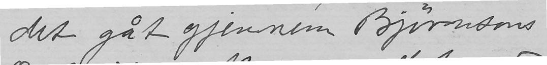det gåt gjennem Bjørnsons
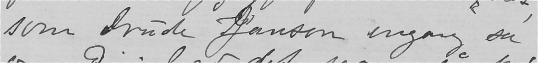som Drude Janson engang sa
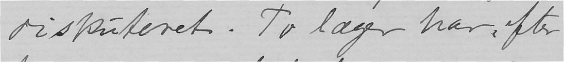diskuteret. To læger har, efter
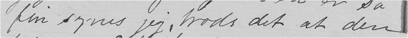fin synes jeg, trods det at den
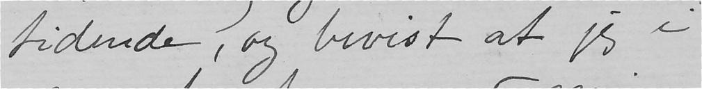tidende, og bevist at jeg i
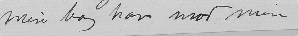min bog han mod min
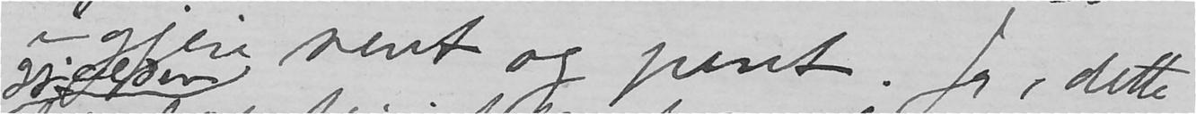igjen rent og pent. Ja, dette
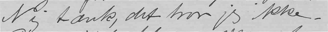Nej tænk, det tror jeg ikke.
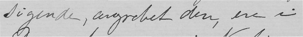sigende, angrebet den, en i
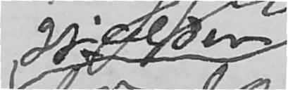gjelder
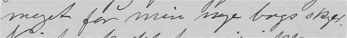meget for min nye bogs skyld.
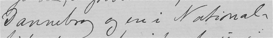Dannebrag og en i National-
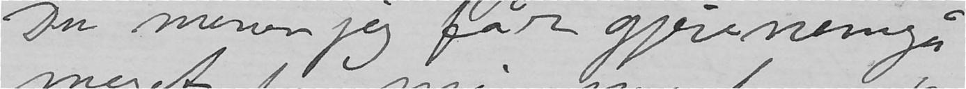Du mener jeg får gjennemgå
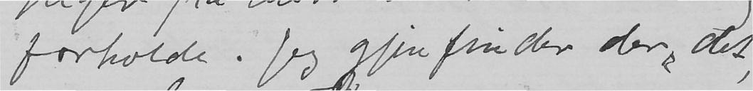forholde. Jeg gjenfinder der,det,
